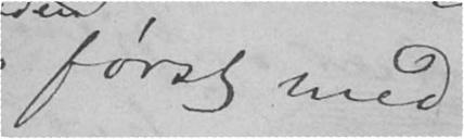først med
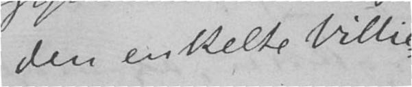den enkelte Villie-
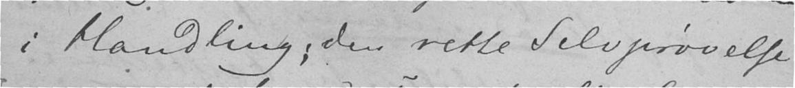i Handling; den rette Selvprøvelse
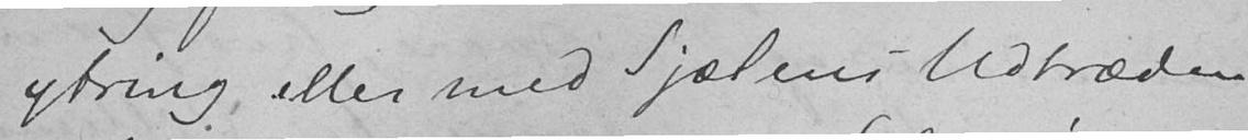ytring eller med Sjælens Udtræden
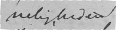neligheden
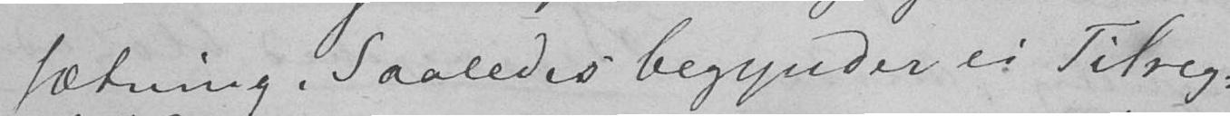sætning. Saaledes begynder ei Tilreg-
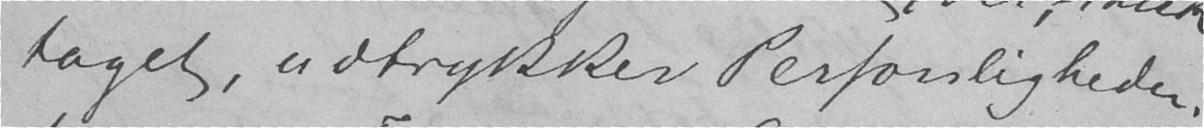taget, udtrykker Personligheden,
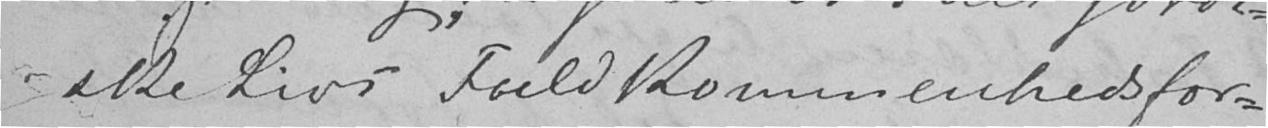ske Livs Fuldkommenhedsfor-
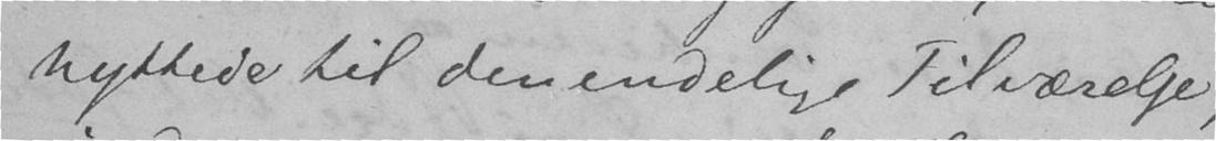nyttede til den endelige Tilværelse,
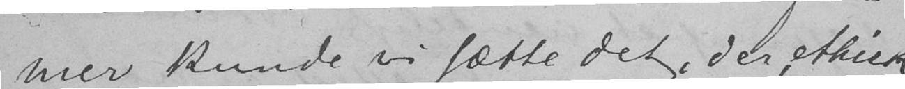mer kunde vi sætte det, der, ethisk
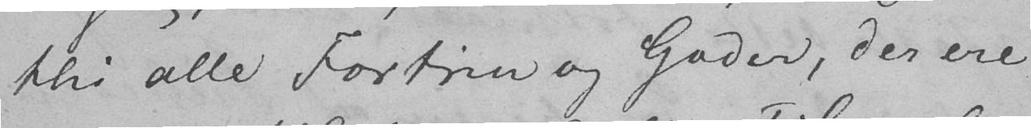thi alle Fortrin og Goder, der ere
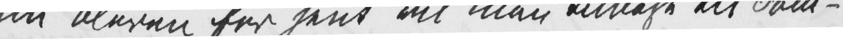nu bleven for sent vil man tilbage til Som¬
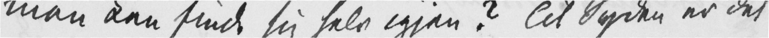man kan finde sig selv igjen? Til Syden er det
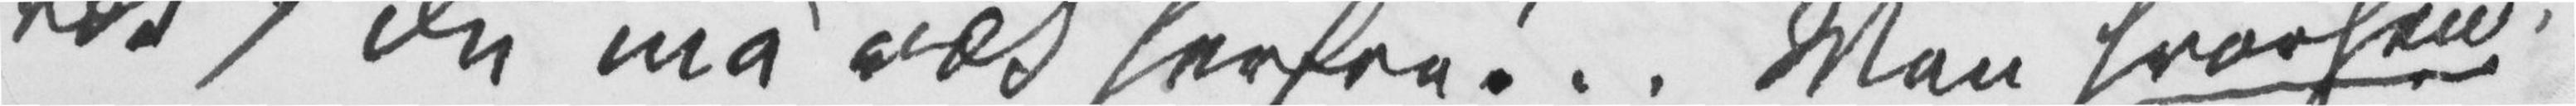væk, du må væk herfra!.. Men hvorhen,
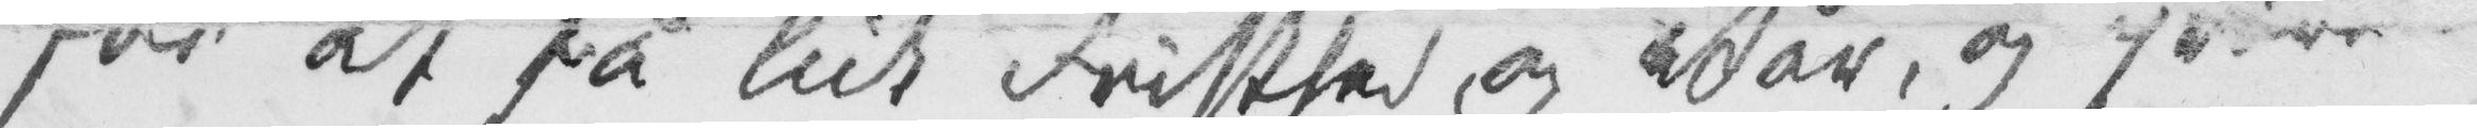for at få lidt Friskhed og Vår, og prøve [om]
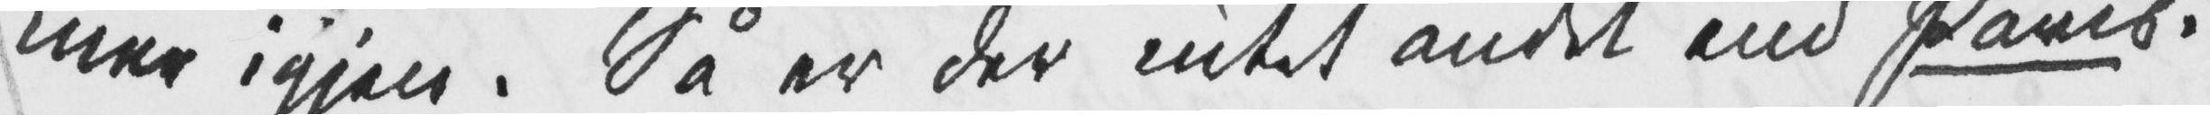mer igjen. Så er der intet andet end Paris.
